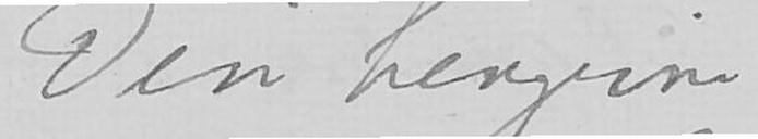Din hengivne
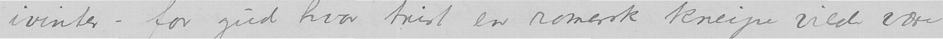ivinter - for gud hvor trist en romersk kneipe vilde være
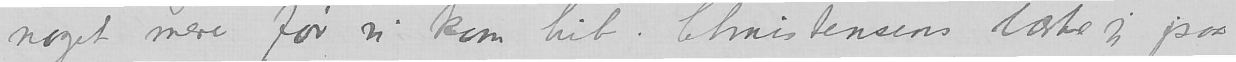noget mere før vi kom hit. Christensens Christensen læste jeg paa
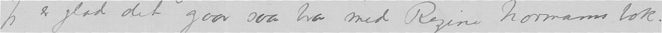Jeg er glad det gaar saa bra med Regine Normanns bok.
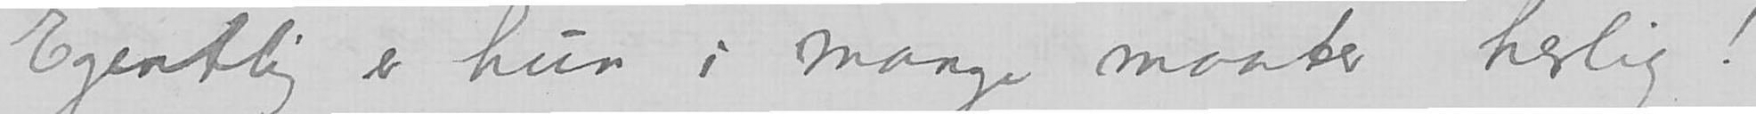Egentlig er hun i mange maater herlig!
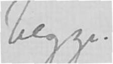begge.
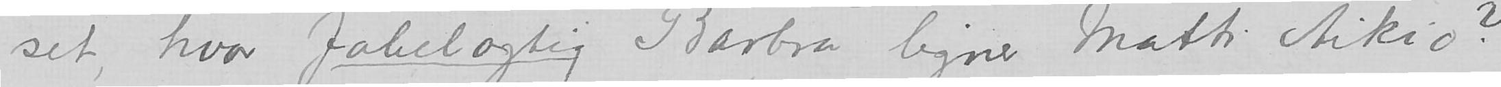set, hvor fabelaktig Barbra ligner Matti Aikio?
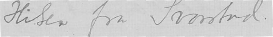Hilsen fra Svarstad
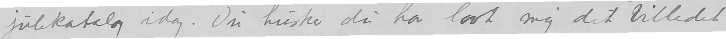julekatalog idag. Du husker du har lovt det billedet.
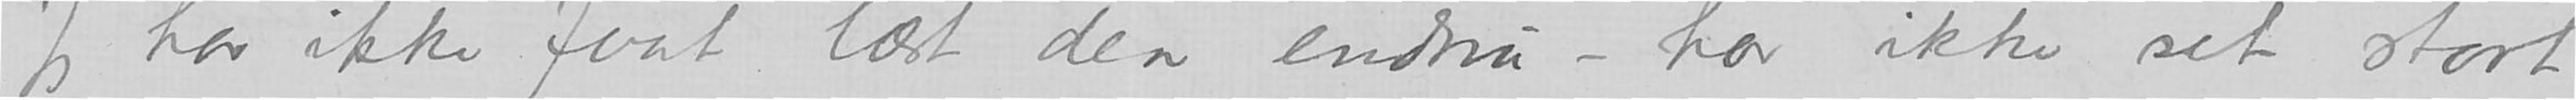Jeg har ikke faat læst den endnu -har ikke set stort
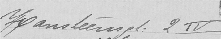Hansteensgt. 2 IV
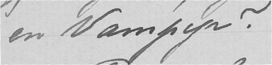en Vampyr?
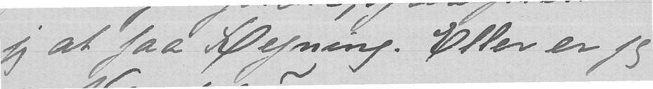jeg at faa Regning. Eller er jeg
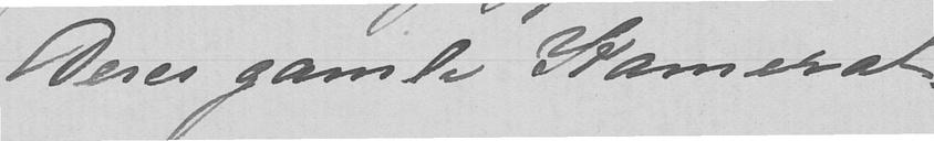Deres gamle Kamerat.
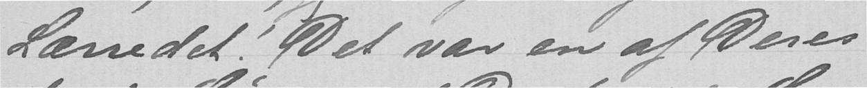Lærredet! Det var en af Deres
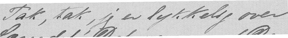Tak, tak, jeg er lykkelig over
